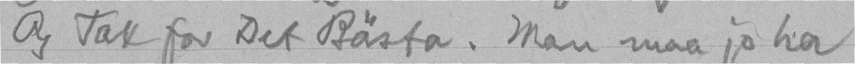Og Tak for Det Bästa. Man maa jo ha
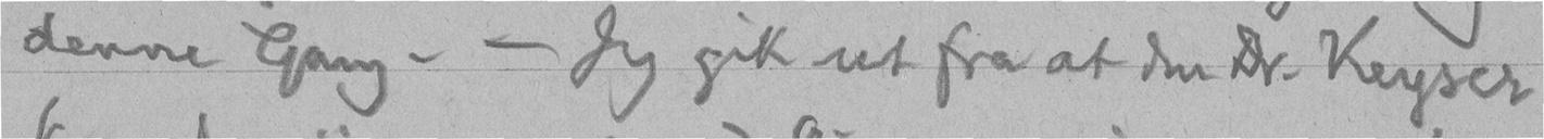denne Gang. - Jeg gik ut fra at den Dr. Keyser
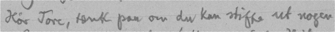Hør Tore, tænk paa om du kan stifte ut nogen
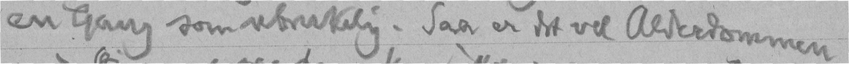en Gang som ubrukelig. Saa er det vel Alderdommen
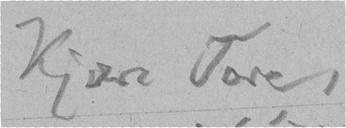Kjære Tore,
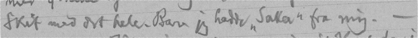Skit med det hele. Bare jeg hadde "Saka" fra mig. -
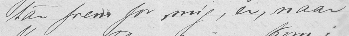tar frem for mig, er, naar
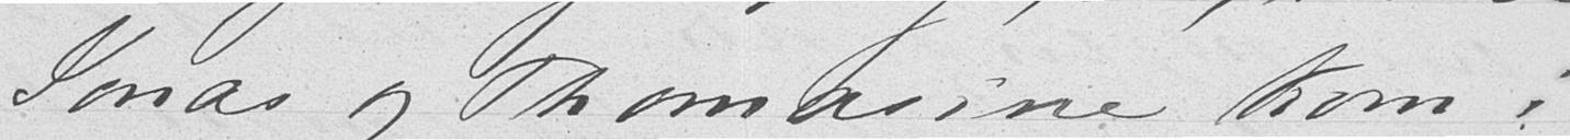Jonas og Thomasine kom i
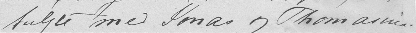fulgte med Jonas og Thomasine.
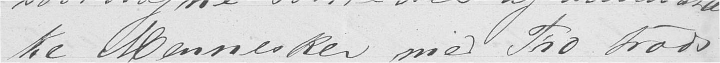ke Mennesker med Tro trods
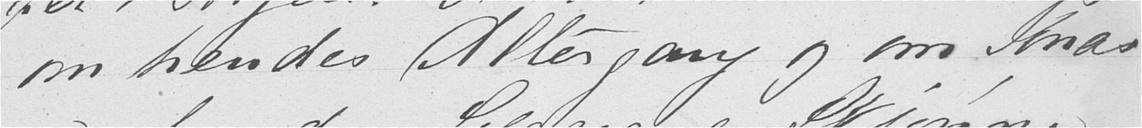om hendes Altergang og om Jonas
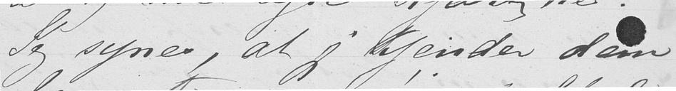Jeg synes, at jeg kjender dem
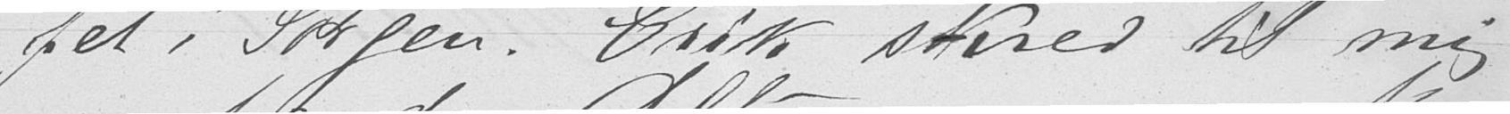pet i Sorgen. Erik skrev til mig
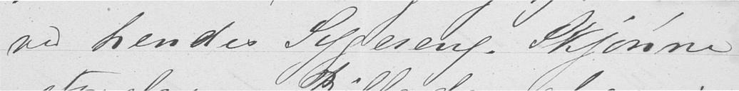ved hendes Sygeseng. Skjønne
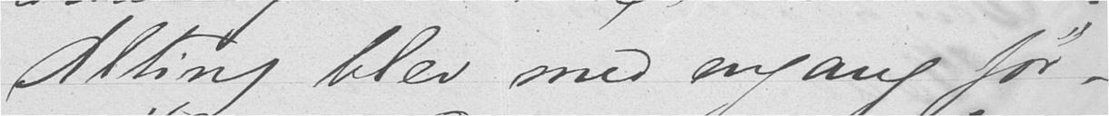Alting blev med engang før-
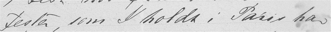Fester, som I holdt i Paris har
